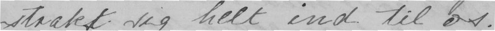strakt sig hel ind til os.
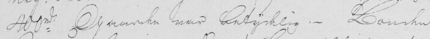400 rde Gaarden var betydelig. - Bonden
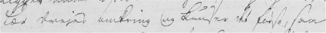lær dreies omkring og knuser det først, saa
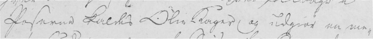Poserne kaldes Olie-Kager, og udgiør en meg-
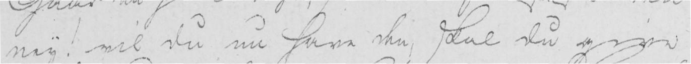ney! vil du nu have den, skal du give
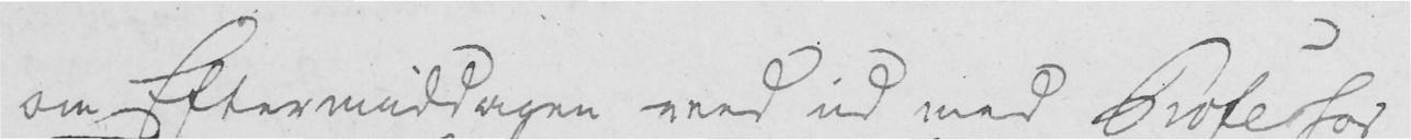om Eftermiddagen red ud med Professor
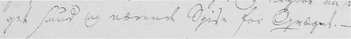get sund og nærende Spise for Qvæget. -
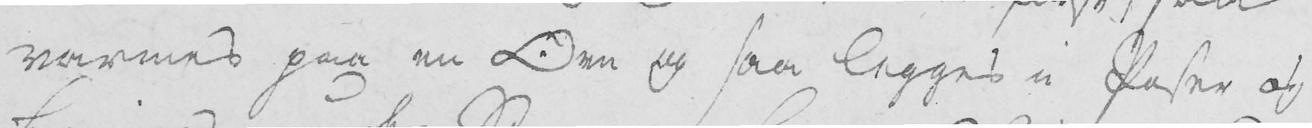varmes paa en Ovn og saa legges i Poser og
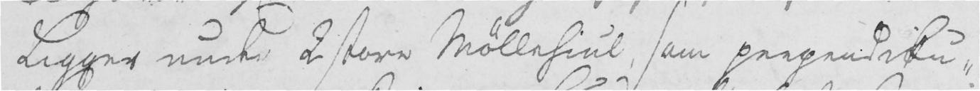ligger under 2 store Møllehiul, som perpendiku-
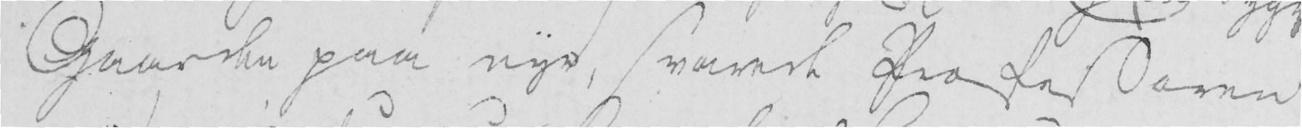Gaarden paa nye, svarede Præste Sønen
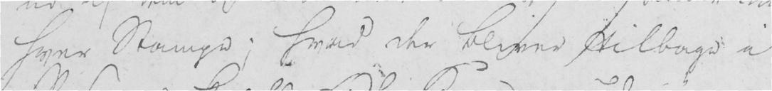hver Stampe; hvad der bliver tilbage i
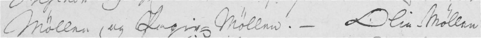Møllen og Papir-Møllen. Olie-Møllen
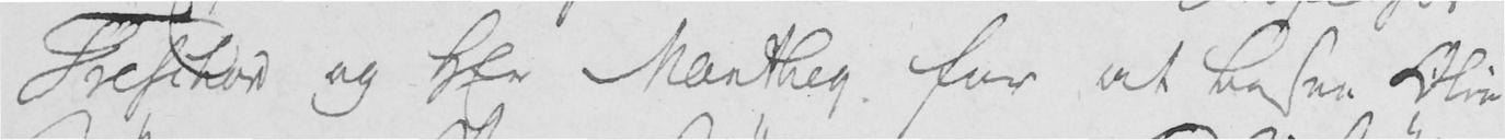Treschow og Hr Manthey for at besee Olie
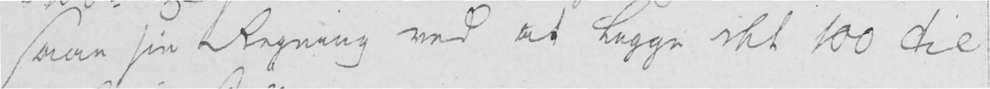saae sin Regning ved at legge det 100 til
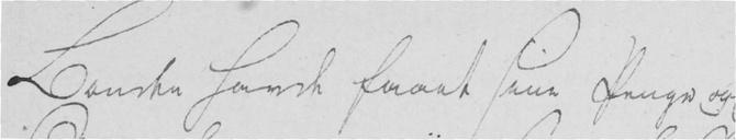Bonden havde faaet sine Penge og
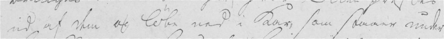ud af dem og løbe ned i Kar, som staaer under
